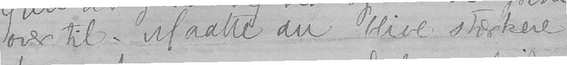over til. Maatte du blive stærkere
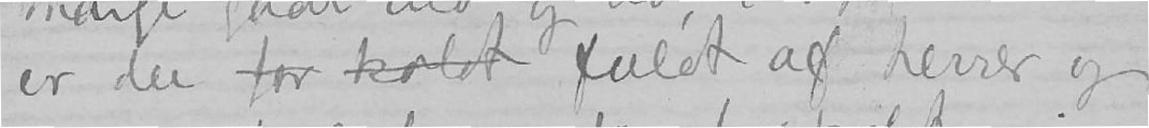er det for koldt fuldt af herrer og
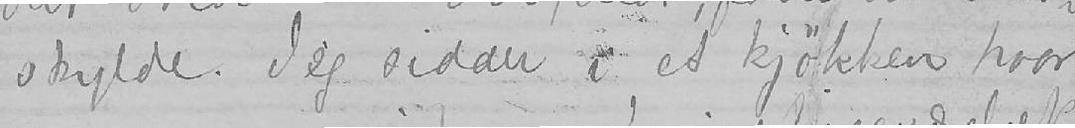skylde. Jeg sidder i et kjøkken hvor
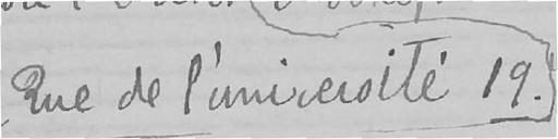Rue de l'université 19.
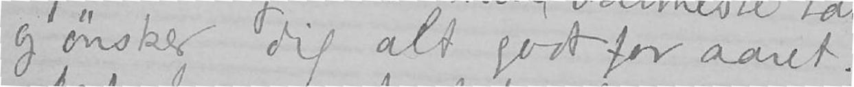og ønsker dig alt godt for aaret.
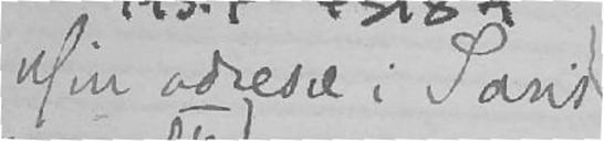Min adresse i Paris
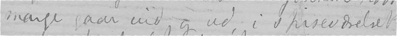mange gaar ind og ud, i spiseværelset
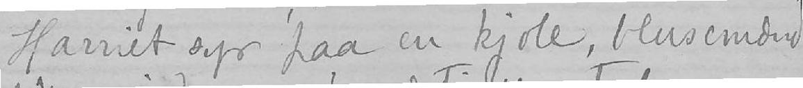Harriet syr paa en kjole, blusemænd
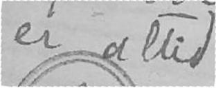er altid
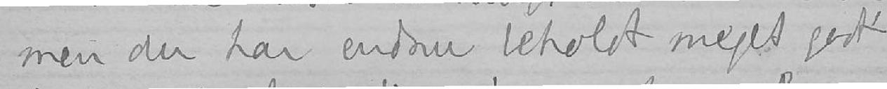men du har endnu beholdt meget godt
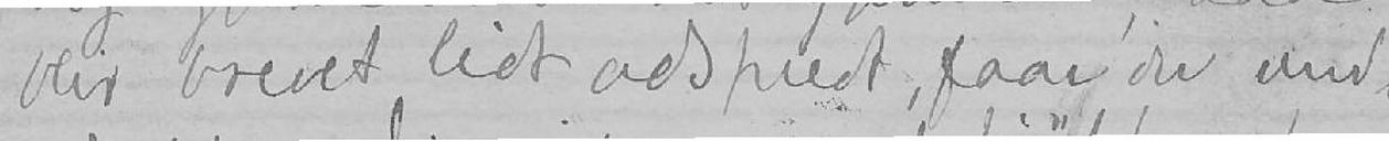blir brevet lidt adspredt, faar du und-
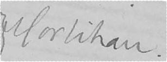Morbihan
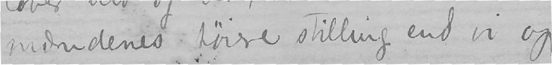mændenes høiere stilling end vi og
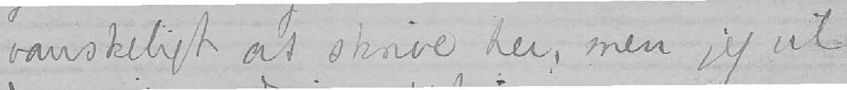vanskeligt at skrive her, men jeg vil
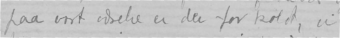paa vort værelse er der for koldt, vi
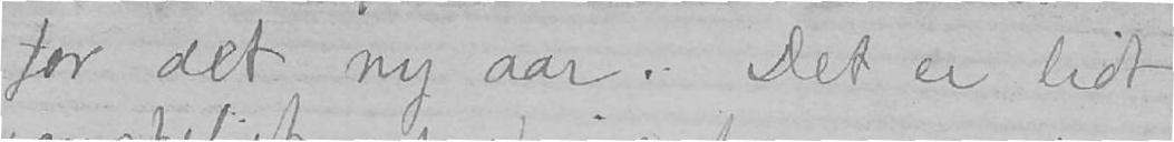for det ny aar. Det er lidt
